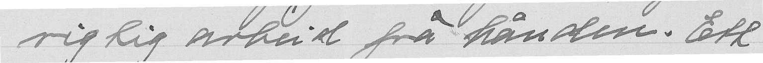rigtig arbeid fra hånden. Ett
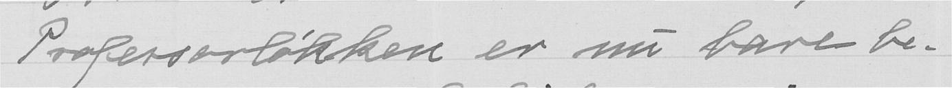Professorløkken er nu bare be-
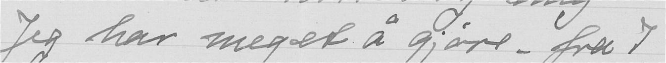Jeg har meget å gjøre - fra 7
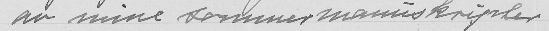av mine sommermanuskripter
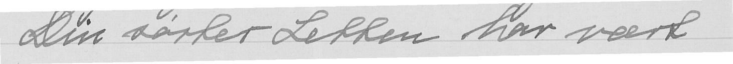Din søster Letten har vært
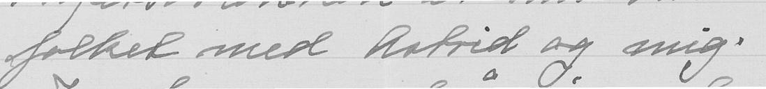folket med Astrid og mig.
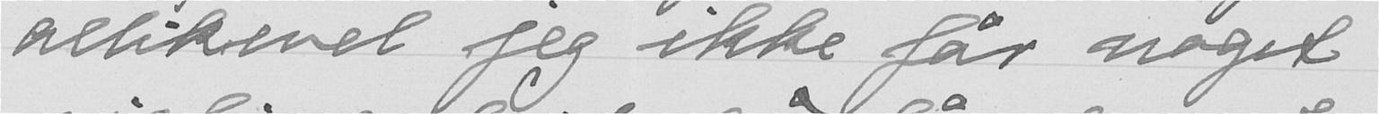allikevel jeg ikke får noget
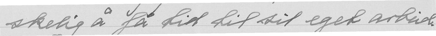skelig å få tid til sit eget arbeid.
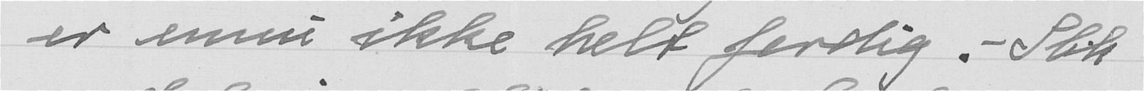er ennu ikke helt ferdig. - Slik
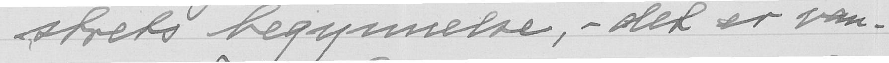strets begynnelse, - det er van-
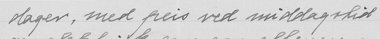dager, med peis ved middagstid
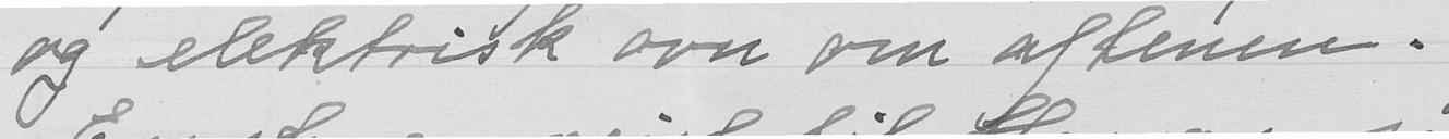og elektrisk ovn om aftenen.
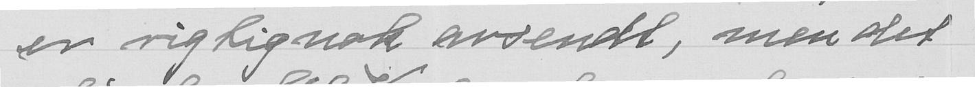er rigtignok avsendt, men det
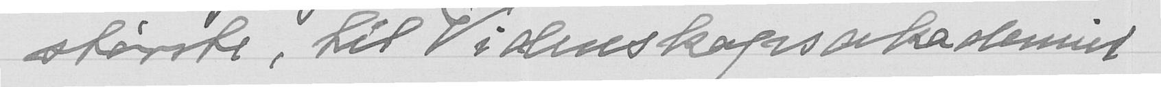største, til Videnskapsakademiet
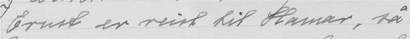Ernst er reist til Hamar, så
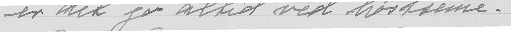er det jo altid ved høstseme-
